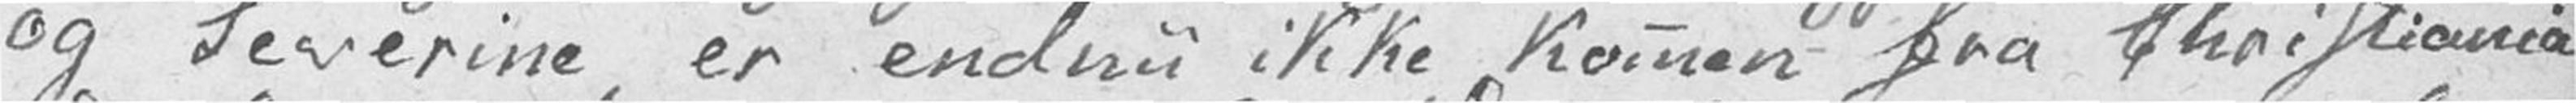og Severine er endnu ikke kommen fra Christiania.
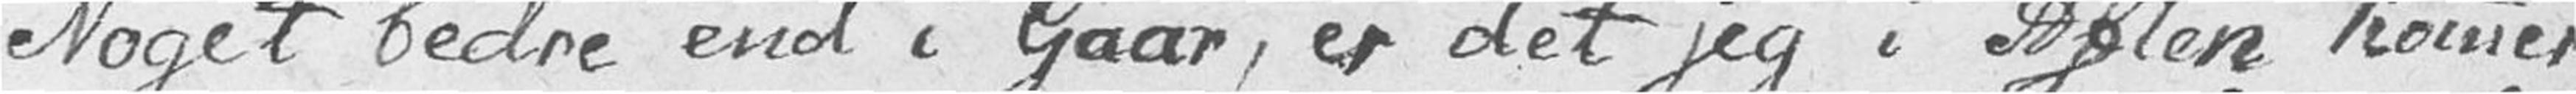Noget bedre end i Gaar, er det jeg i Aften kommer
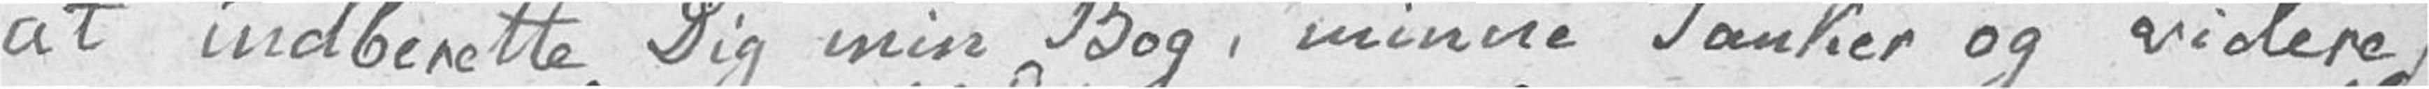at indberette Dig min Bog, minne Tanker og videre,
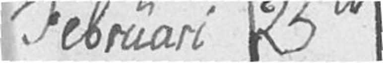Februari 25d
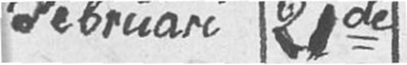Februari 21de
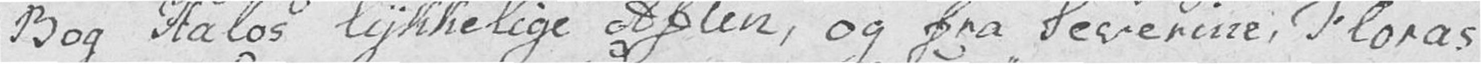Bog Halos lykkelige Aften, og fra Severine, Floras
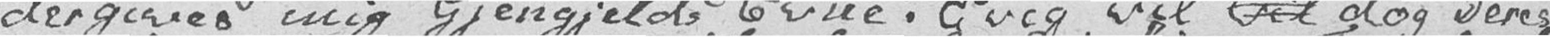der gives mig Gjengjelds Evne. Evig vil vil dog Deres
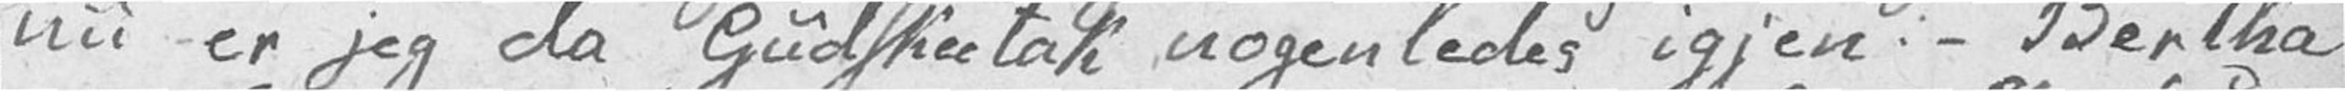nu er jeg da Gudskeetak nogenledes igjen. – Bertha
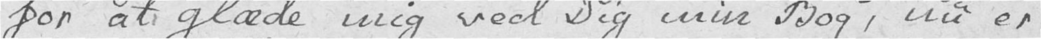for at glæde mig ved Dig min Bog, nu er
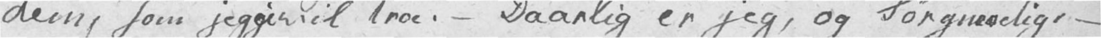dem, som jeg gir vil troe. – Daarlig er jeg, og Sørgmodig. –
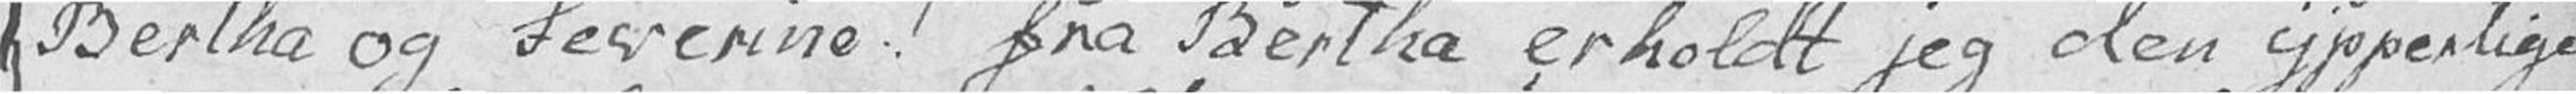Bertha og Severine! fra Bertha erholdt jeg den ypperlige
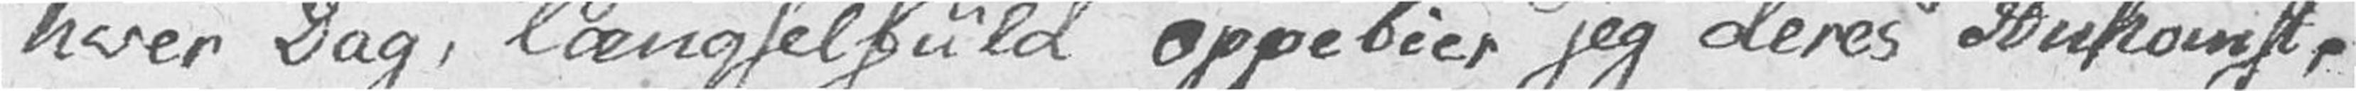hver Dag, længselfuld oppebier jeg deres Ankomst.
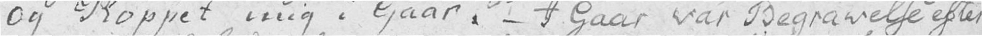og Koppet mig i Gaar. – I Gaar var Begravelse efter
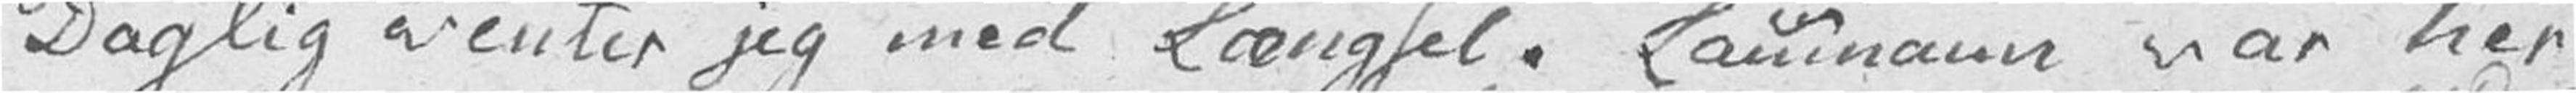Daglig venter jeg med Længsel. Laumann var her
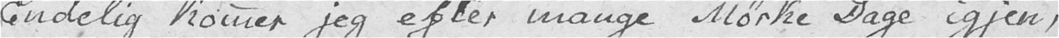Endelig kommer jeg efter mange Mørke Dage igjen,
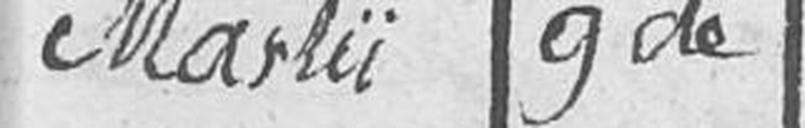Martii 9de
